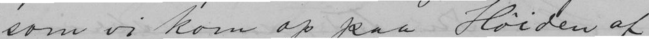som vi kom op paa Høiden af
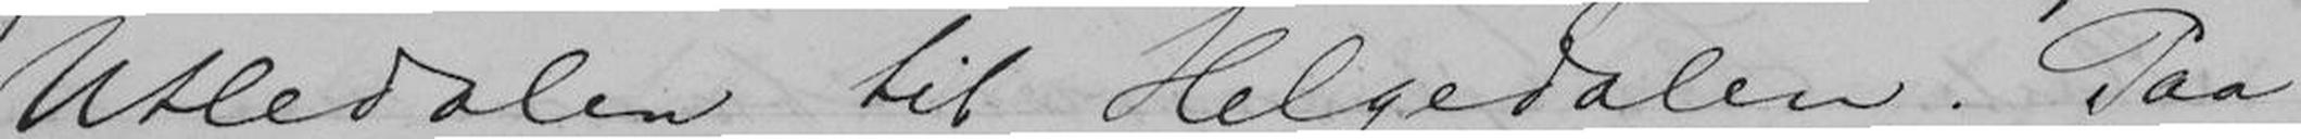Utledalen til Helgedalen. Paa
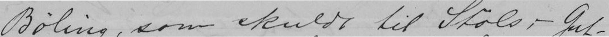Bøling, som skulde til Støls, - Gut-
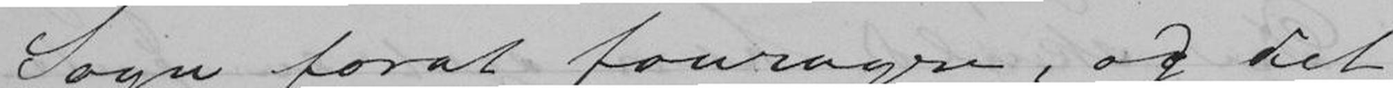Sogn forat fouragere, og det
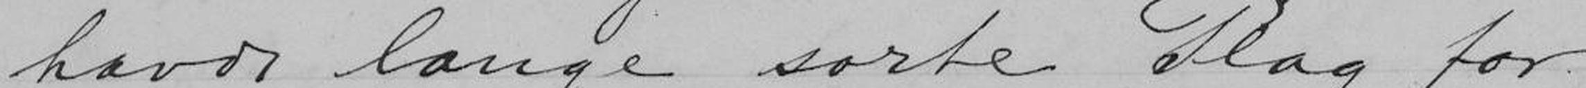havde lange sorte Plag for
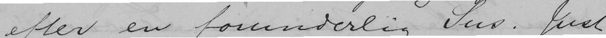efter en forunderlig Sus. Just
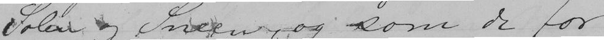Solen og Sneen, og som de for
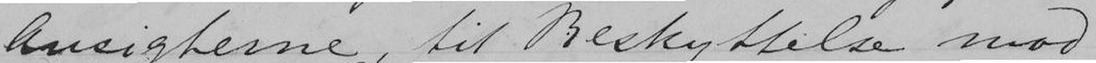Ansigterne, til Beskyttelse mod
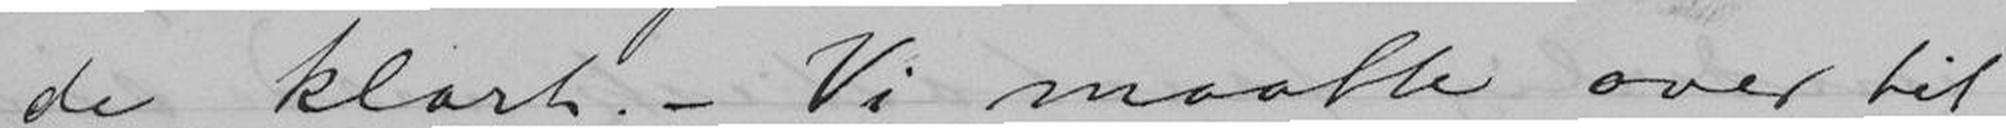de klart. - Vi maatte over til
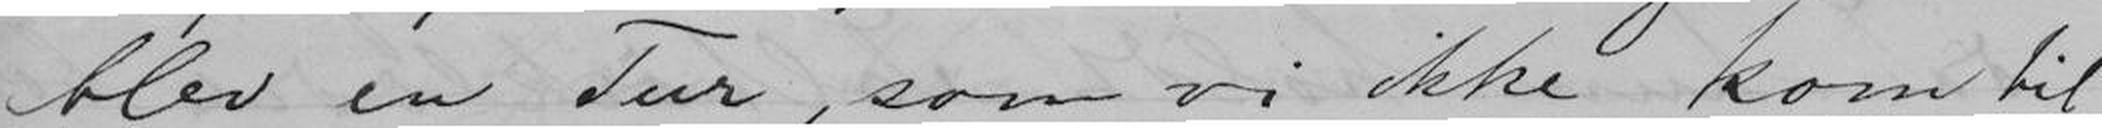blev en Tur, som vi ikke kom til
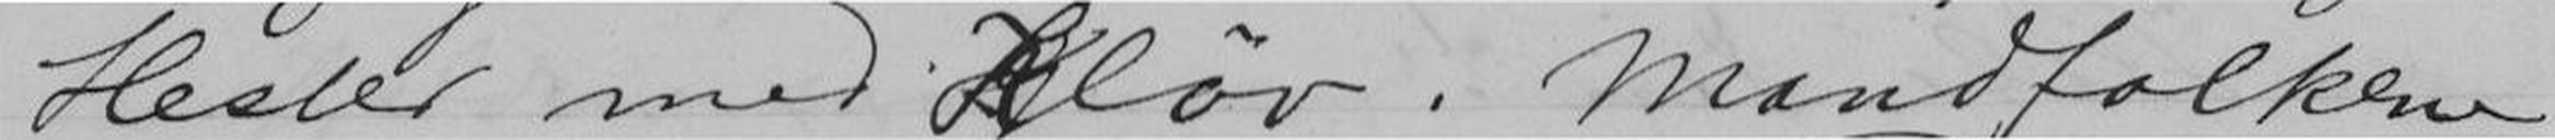Hester med Kløv. Mandfolkene
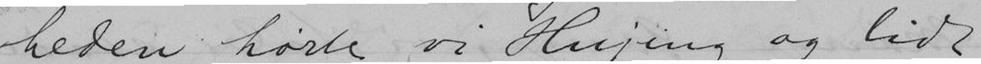heden hørte vi Hujing og lidt
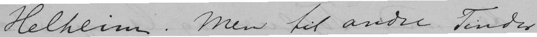Helheim. Men til andre Tinder
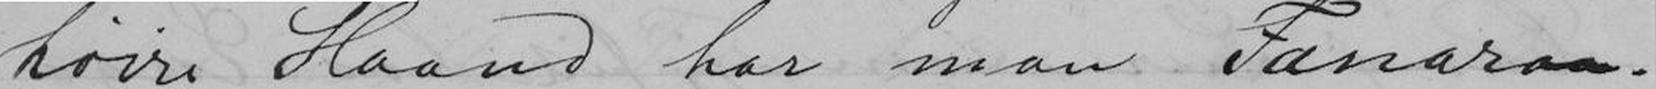høire Haand har man Fanaraa-
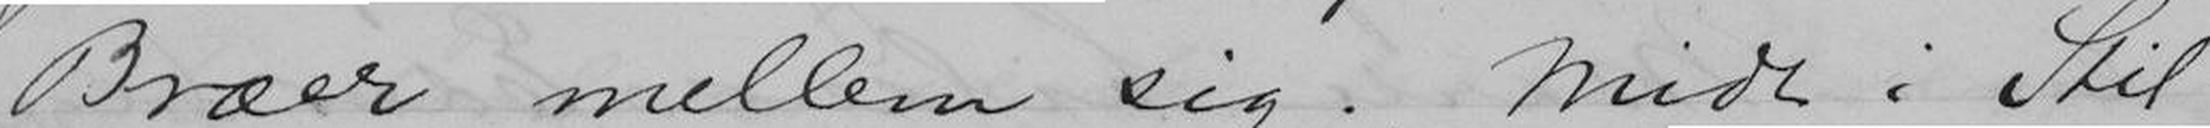Bræer mellem sig. Midt i Stil-
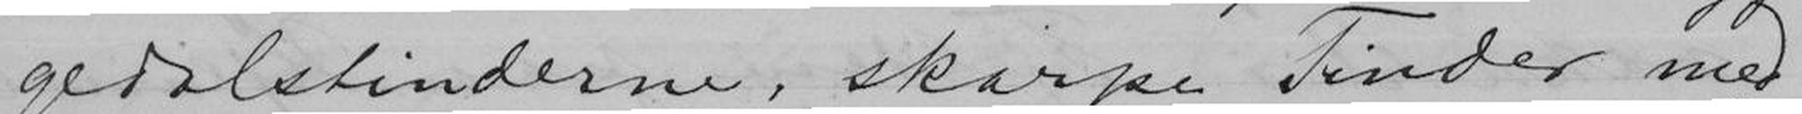gedalstinderne, skarpe Tinder med
